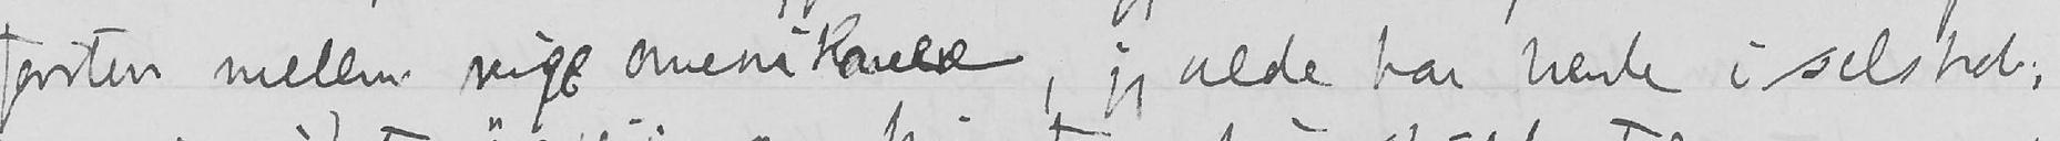farten mellem rige amerikanere, jeg vilde have hende i selskab,
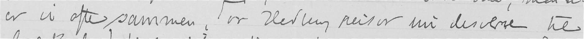er vi ofte sammen, Tor Hedberg reiser nu desverre til
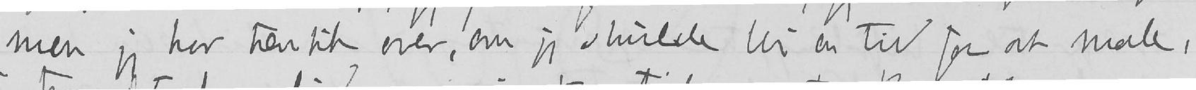men jeg har tænkt over, om jeg skulde bli en tid for at male,
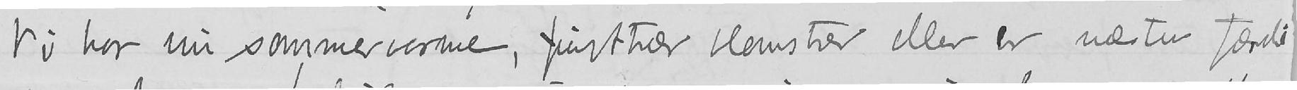Vi har nu sommervarme, frugttrær blomstrer eller er næsten færdi-
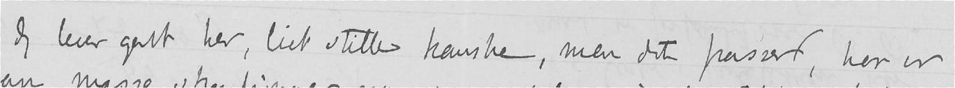Jeg lever godt her, lidt stille kanske, men det passer, her er
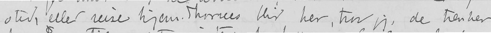sted, eller reise hjem. Thornes blir her, tror jeg, de tænker
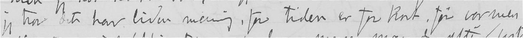jeg tror det har liden mening, for tiden er for kort, før varmen
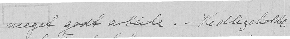meget godt arbeide. - Vedligeholdel-
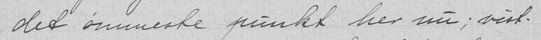det ømmeste punkt her nu; vist-
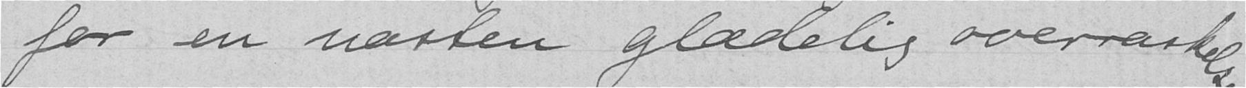for en næsten glædelig overraskelse.
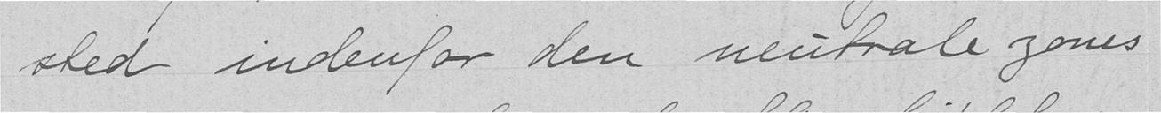sted indenfor den neutrale zones
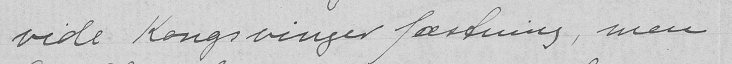vide Kongsvinger fæstning, men
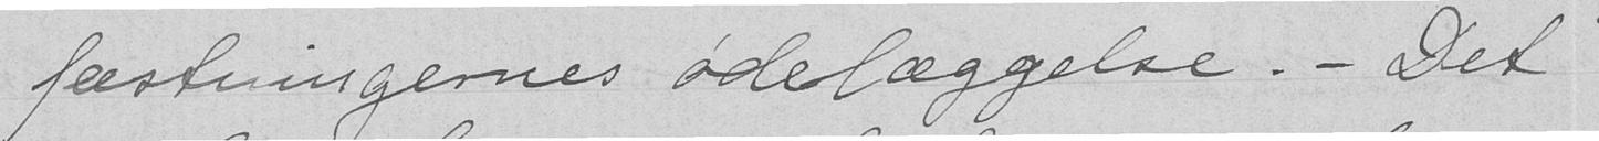fæstningernes ødelæggelse. - Det
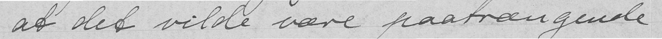at det vilde være paatrængende
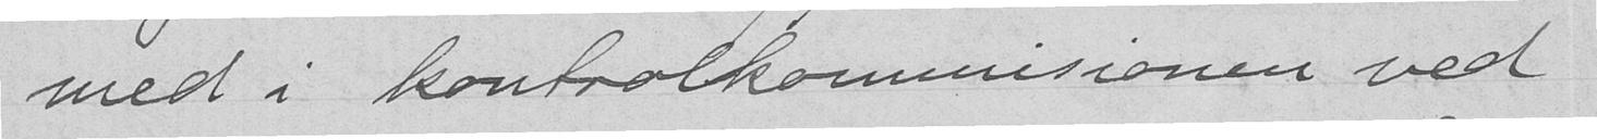med i kontrolkommisionen ved
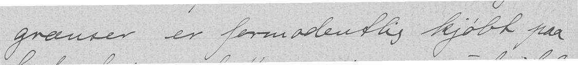grænser er formodentlig kjøbt paa
